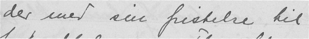der med sin fristelse til
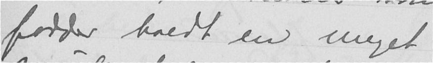fadder holdt en meget
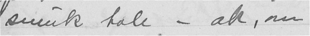smuk tale - ak, om
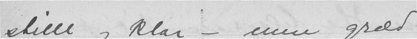stille og klar - men græd
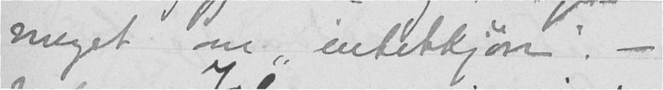meget om "intetkjøn". -
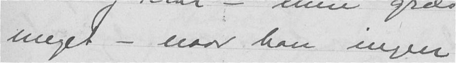meget - naar han ingen
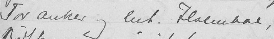Tor Anker og lnt. Holmboe,
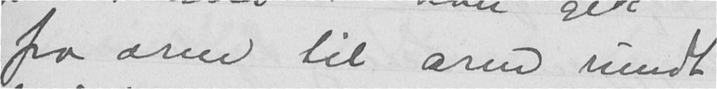fra arm til arm rundt
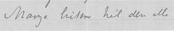Mange hilsener til dere alle
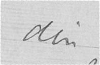din
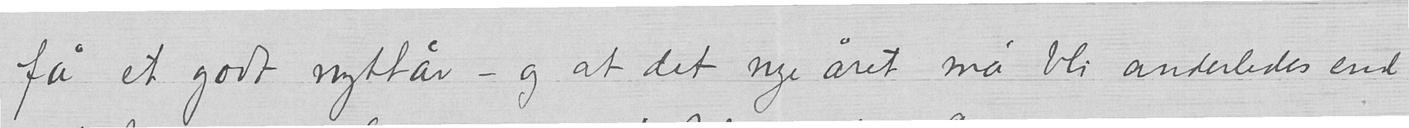få et godt nyttår – og at det nye året må bli anderledes end
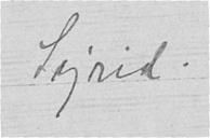Sigrid.
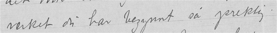værket du har begynnt så prektig.
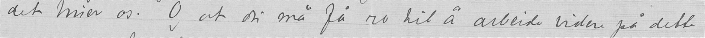det truer os. Og at du må få ro til å arbeide videre pa dette
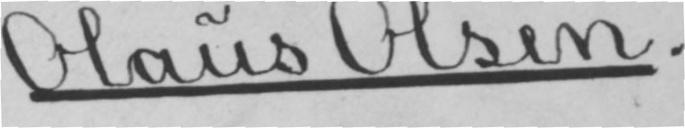Olaus Olsen.
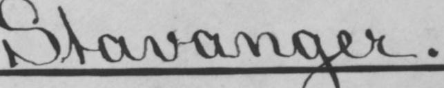Stavanger.
